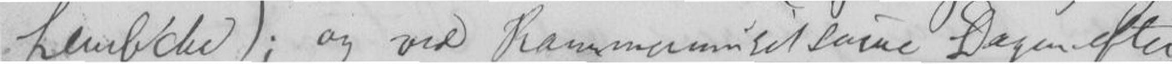Lambche); og ved Kammermusiksoire Dagen efter
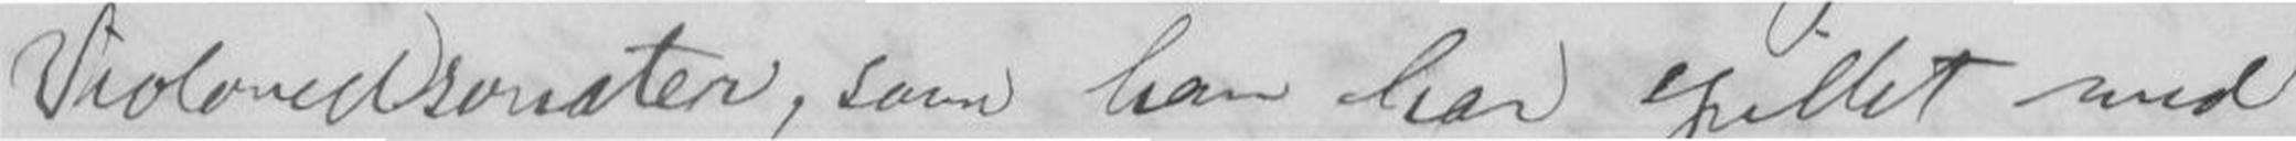Violonellsonater, som han har spillet med
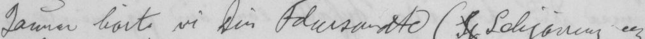Januar hørte vi Din Fdursonate (Sj Schjöning og
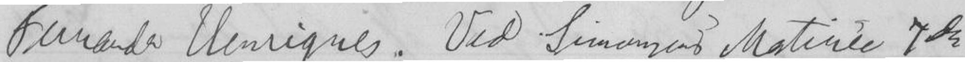Fernanda Henriques. Ved Simonsens Matinée 7de
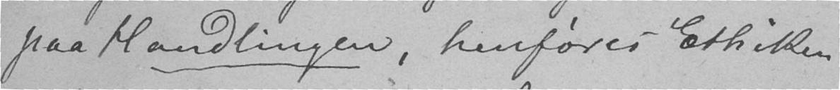paa Handlingen, henføres Ethiken
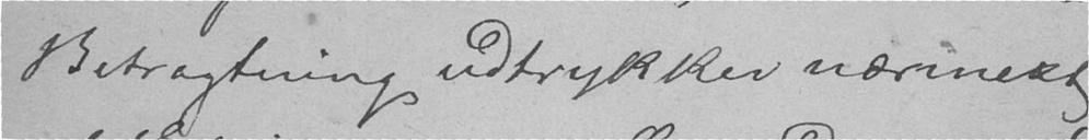Betragtning udtrykker nærmest,
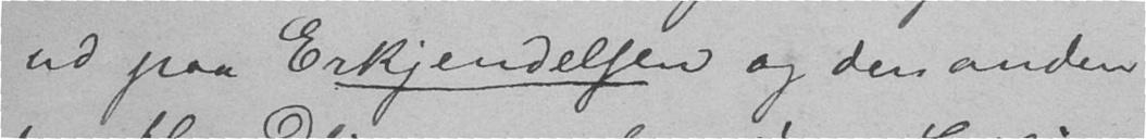ud paa Erkjendelsen og den anden
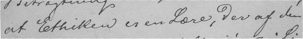at Ethiken er en Lære, der af den
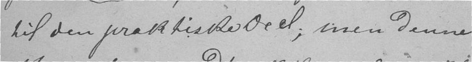til den praktiske Deel, men denne
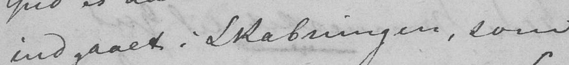indgaaet i Skabningen, som
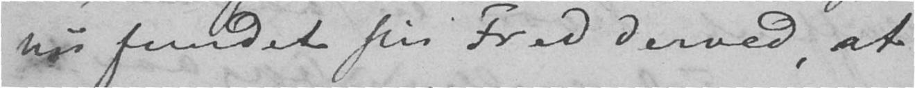nu fundet sin Fred derved, at
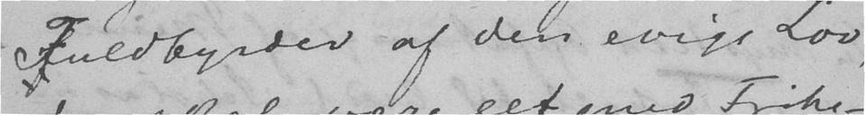Fuldbyrder af den evige Lov,
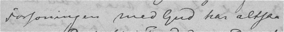Forsoningen med Gud har altsaa
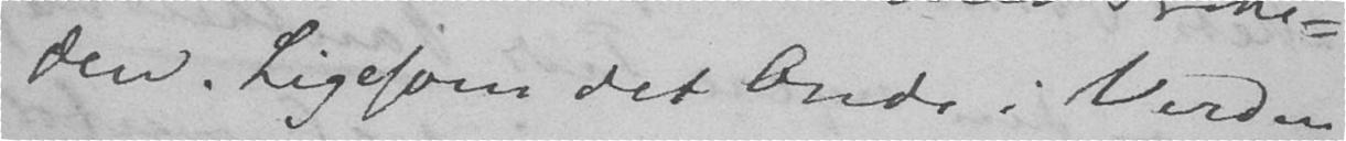den. Ligesom det Onde i Verden
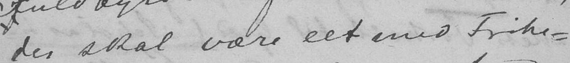der skal være eet med Frihe-
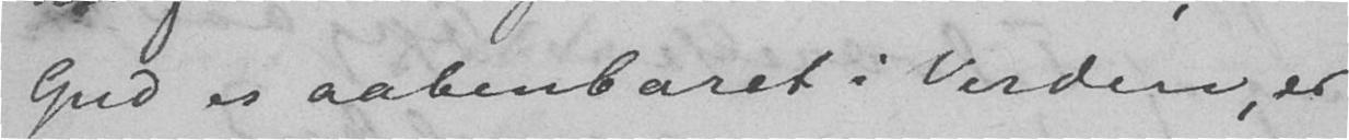Gud er aabenbaret i Verden, er
